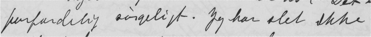forfærdelig sørgeligt. Jeg har slet ikke
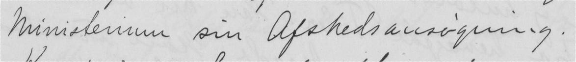ministerium sin Afskedsansøgning.
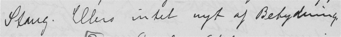Stang. Ellers intet nyt af Betydning.
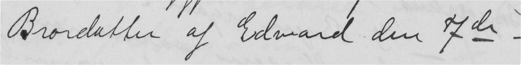Brordatter af Edvard den 7de
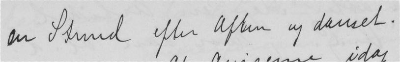en Stund efter Aften og danset.
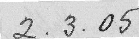2.3.05
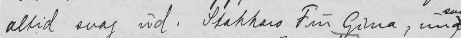altid svag ud. Stakkars Fru Gina, nu
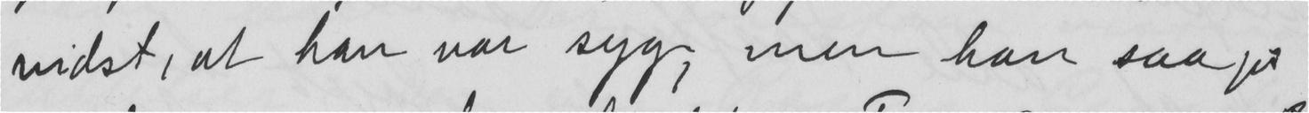vidst, at han var syg, men han saage
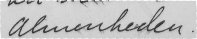Almenheden.
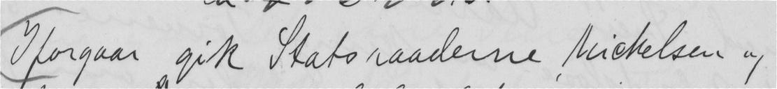Iforgaar gik Statsraaderne Michelsen og
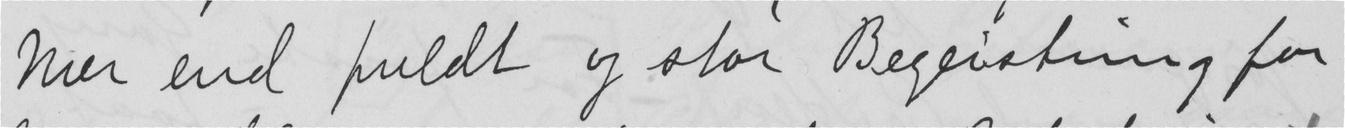Mer end fuldt og stor Begeistring for
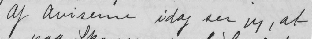af Aviserne idag ser jeg, at
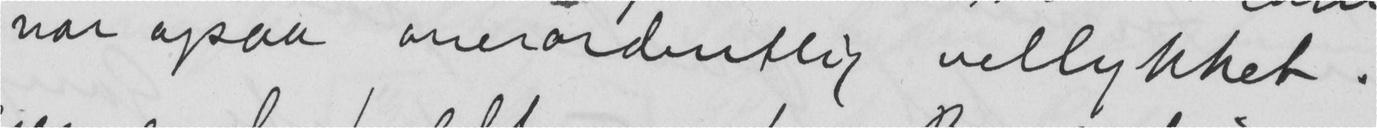var ogsaa overordentlig vellykket.
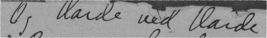Og Varde ved Varde
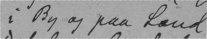i By og paa Land
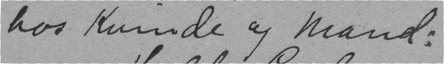hos Kvinde og mand:
